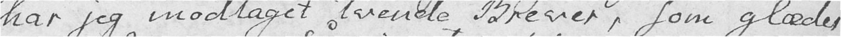har jeg modtaget tvende Brever, som glæder
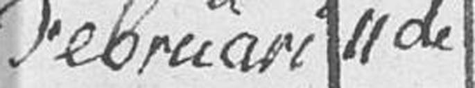Februari 11de
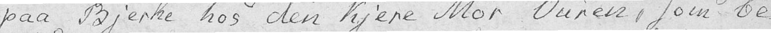paa Bjerke hos den kjere Mor Ouren, som be
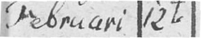Februari 12te
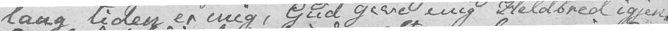lang tiden er mig, Gud give mig Heldbred igjen.
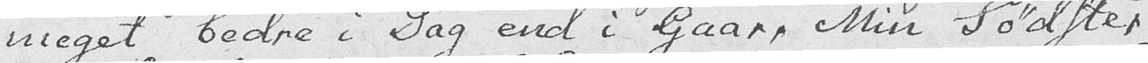meget bedre i Dag end i Gaar. Min Sødster
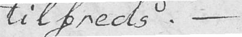tilfreds. –
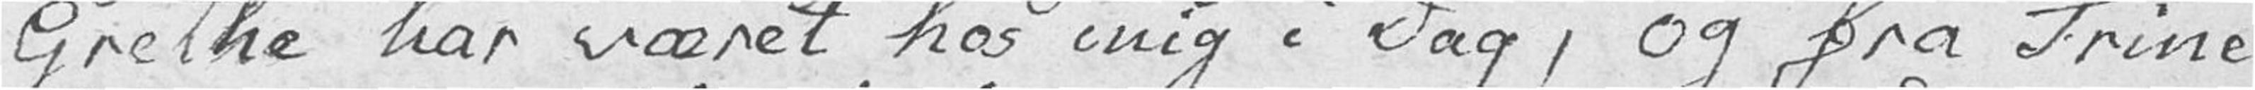Grethe har været hos mig i Dag, og fra Trine
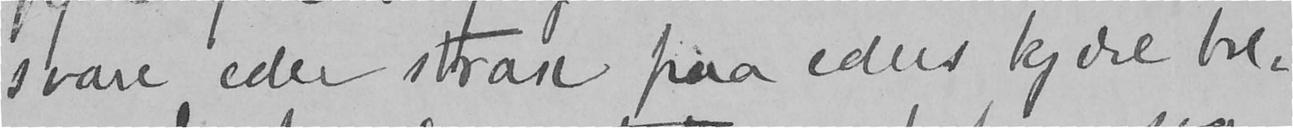svare eder strax paa eders kjære bre-
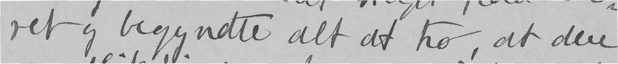ret og begyndte alt at tro, at dere
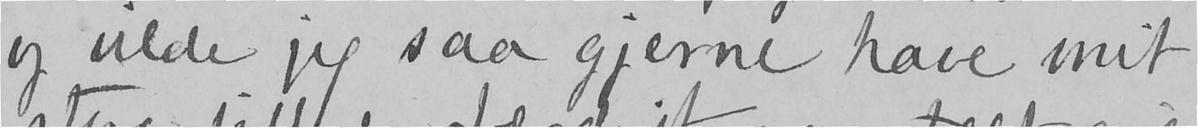og vilde jeg saa gjerne have mit
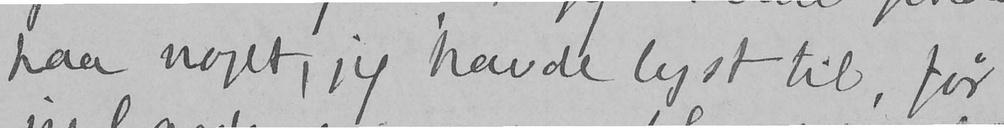paa noget, jeg havde lyst til, før
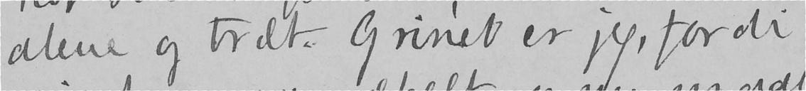alene og træt. Grinet er jeg, fordi
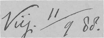Viig. 11/9 88.
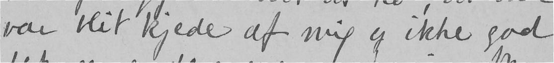var blit kjede af mig og ikke gad
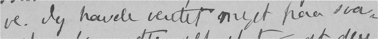ve. Jeg havde ventet meget paa sva-
